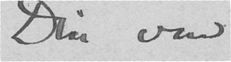Din ven
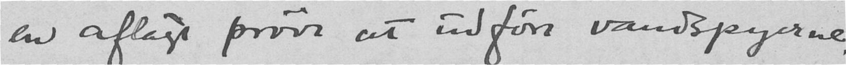en aflagt prøve at udføre vandspyerne.
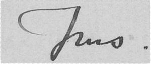Jens.
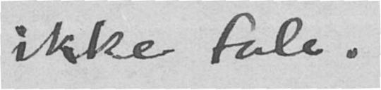ikke tale.
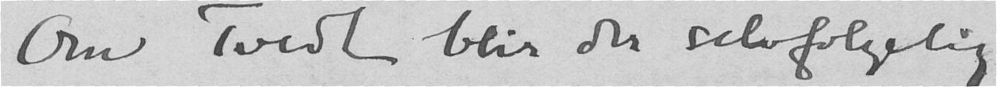Om Tvedt blir der selvfølgelig
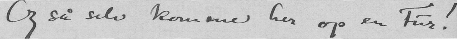Og så selv komme her op en tur!
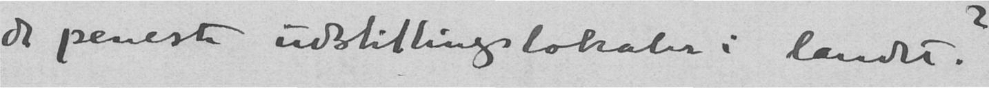de peneste udstillingslokaler i landet?
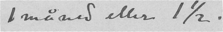1 måned eller 1 1/2.
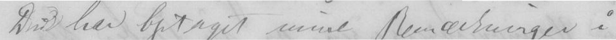Du har Optaget mine Bemærkninger i
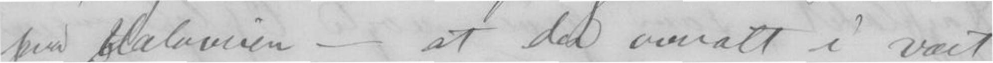paa Halvveien - at der overalt i vort
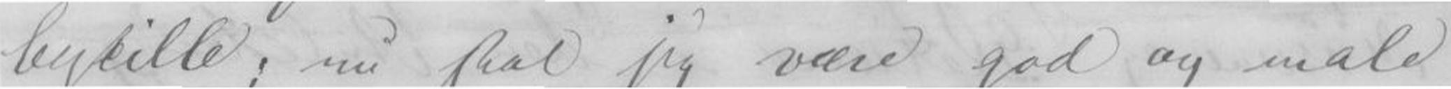bestille; nu skal jeg være god og male
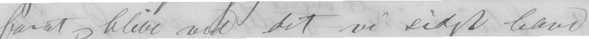forat blive ved det vi sidst have
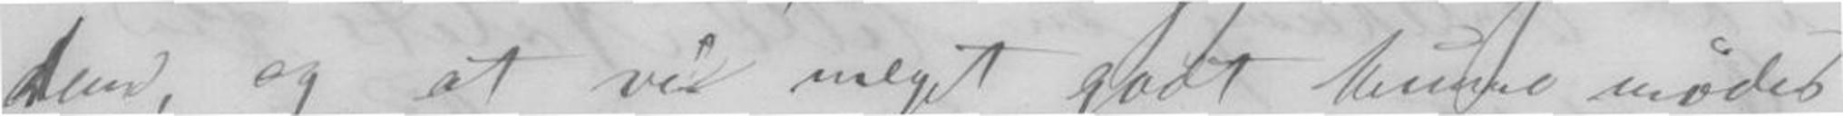dem, og at vi meget godt kunne mødes
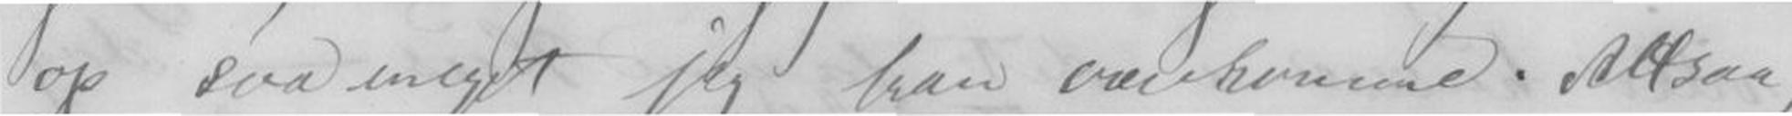op saa meget jeg kan overkomme Altsaa,
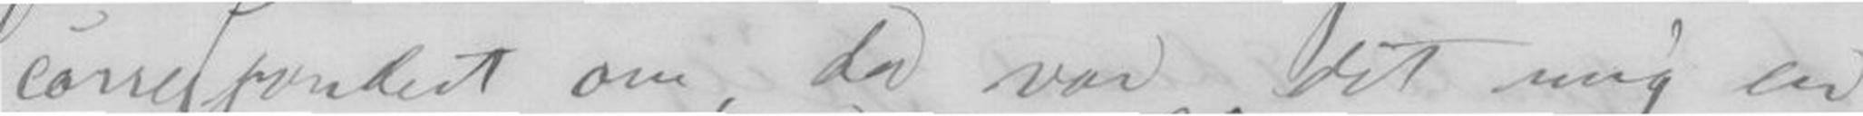correspondert om, da var det mig en
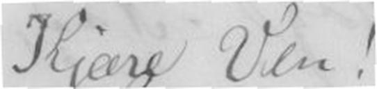Kjære Ven!
